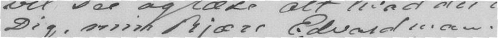Dig, min kjære Edvardman.
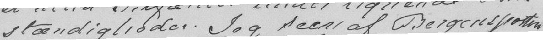stændigheder. Jeg seer af Bergensposten
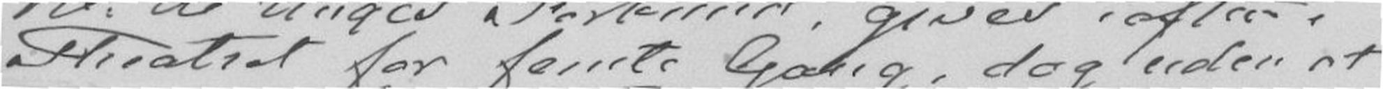Theatret for fente Gang, dog uden at
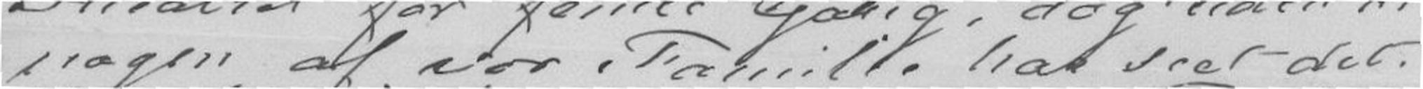nogen af vor Familie har seet det.
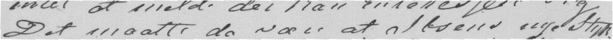Det maatte da være at Ibsens nye Styk-
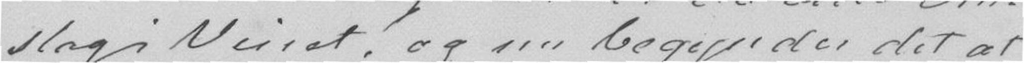slag i Veiret, og nu begynder det at
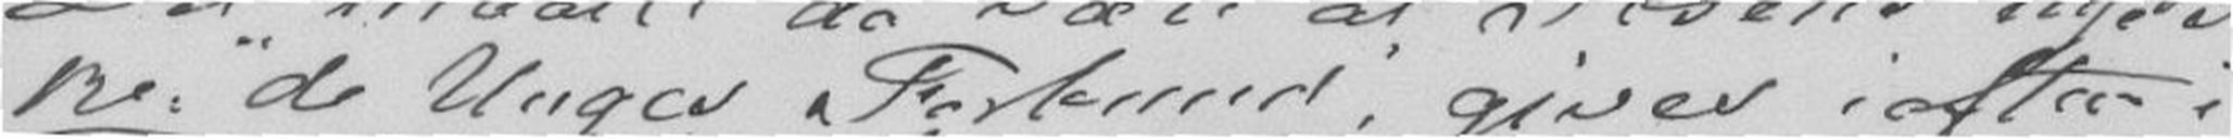ke: "de Unges Forbund", gives iaften i
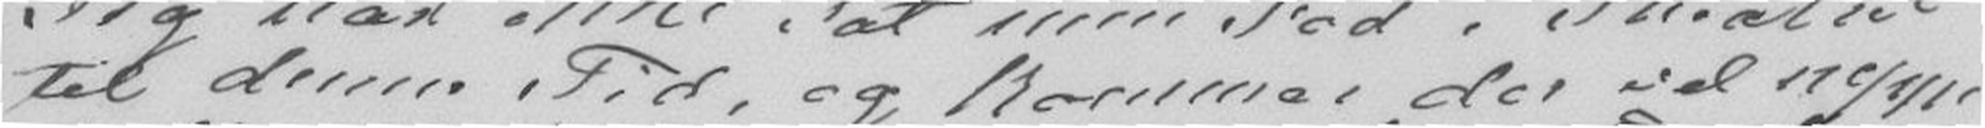til denne Tid, og kommer der vel neppe
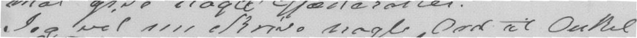Jeg vil nu skrive nogle Ord til Onekel
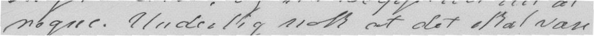regne. Underlig nok at det skal være
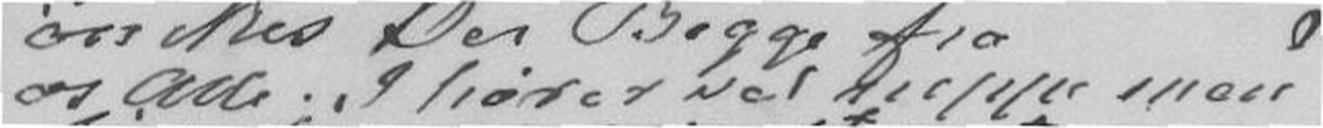os Alle. I hører vel neppe mere
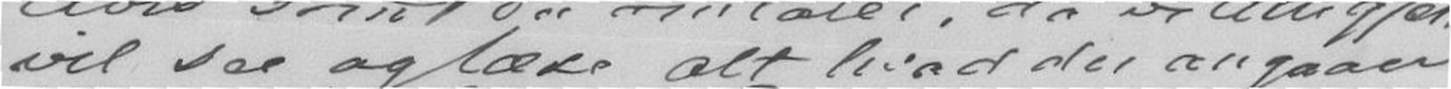vil see og læse alt hvad der angaar
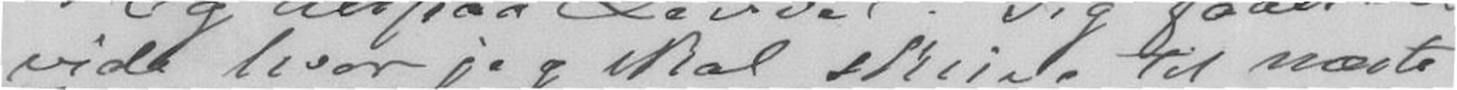vide hvor jeg skal skrive til næste
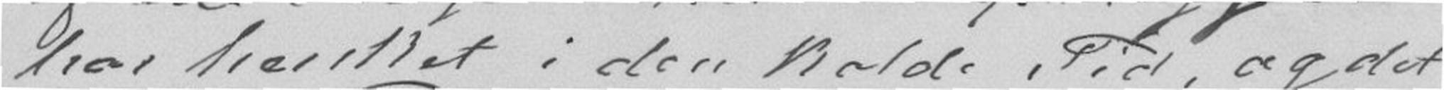har hersket i den kolde Tid, og det
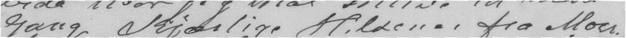Gang Kjærlige Hilsener fra Moer,
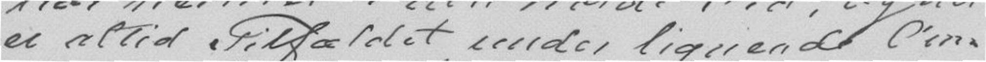er altid Tilfældet under lignende Om-
